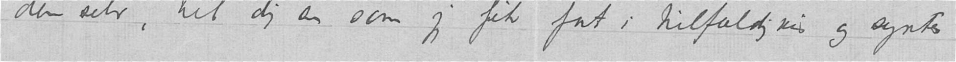den selv, til dig en som jeg fik fat i tilfældigvis og syntes
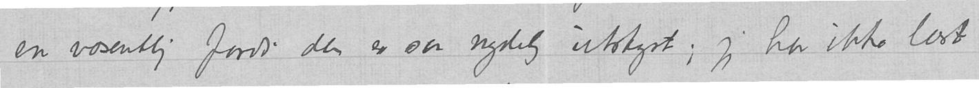en væsentlig fordi den er saa nydelig utstyrt; jeg har ikke læst
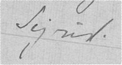Sigrid.
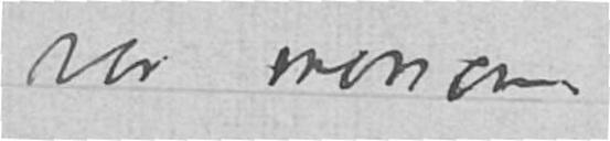var morsom.
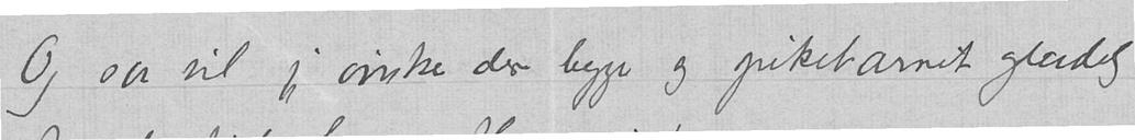Og saa vil jeg ønske dere begge og pikebarnet glædelig
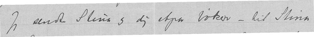Jeg sendte Stina og dig etpar bøker – til Stina
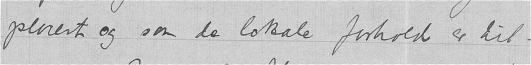placert og som de lokale forhold er til - .
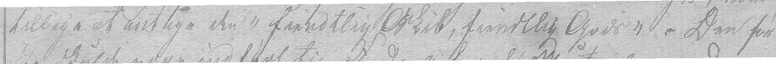tillige at antage den "fiendtlig Skib, fiendtlig Gods". Den før-
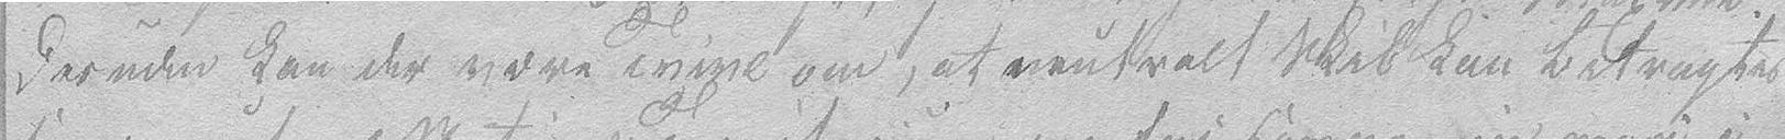Desuden kan der være Tvivl om, at neutralt Skib kan betragtes
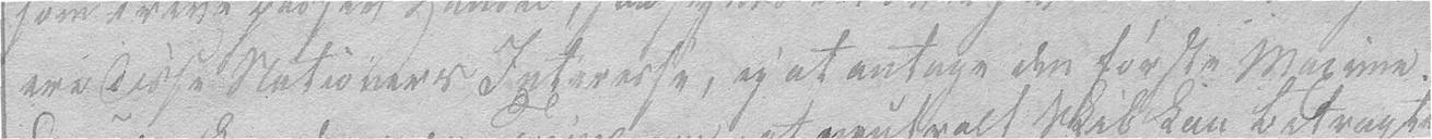ere disse Nationers Interesse, ey at antage den første Maxime.
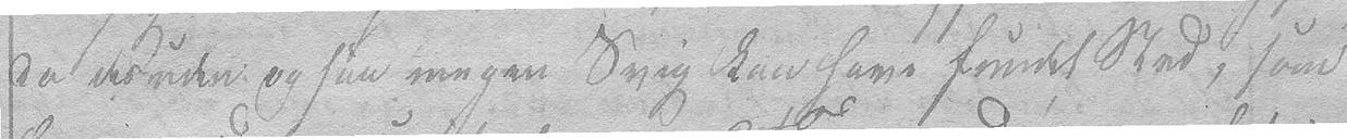da desuden ogsaa megen Svig kan have fundet Sted, som
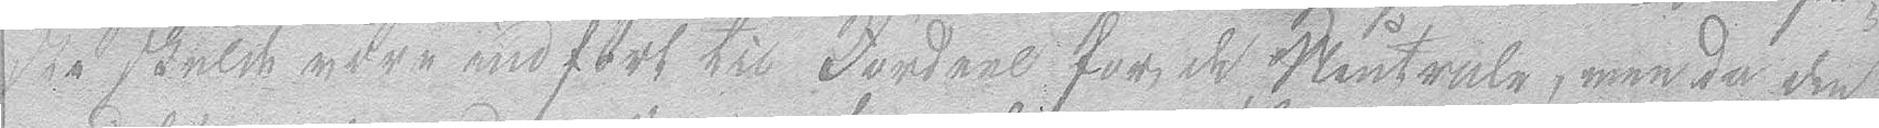ste skulde være indført til Fordeel for de Neutrale, men da den
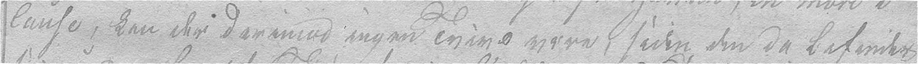cause, kan der derimed ingen Tvivl være, siden den da befinder
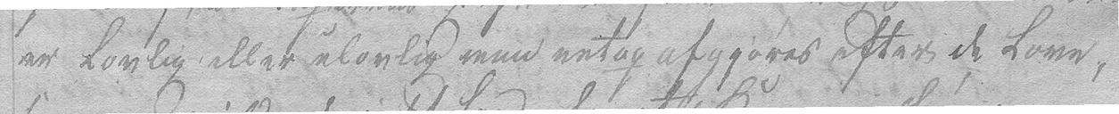er lovlig eller ulovlig maa netop afgjøres efter de Love,
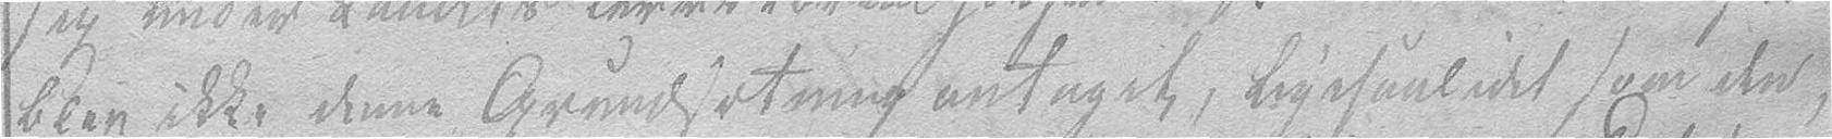blev ikke denne Grundsætning antaget, ligesaalidet som den,
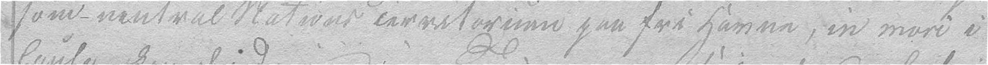som neutral Nations Territorium paa fri Havne, in mori i
